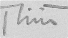Thiis
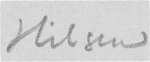Hilsen
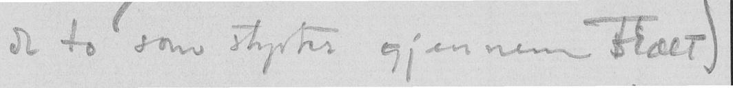de to som styrter gjennem Træet)
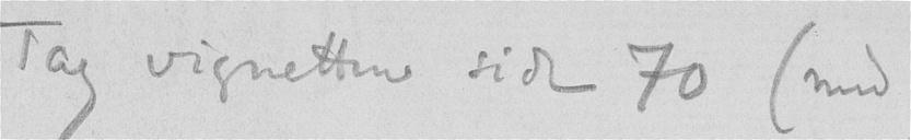Tag vignetten side 70 (med
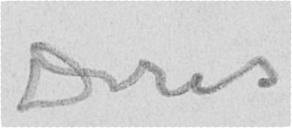Deres
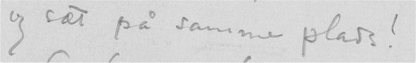og sæt på samme plads!
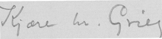Kjære hr. Grieg
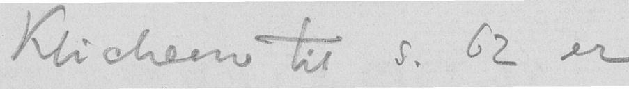Klicheen til s. 62 er
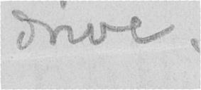drive.
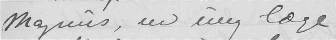Magnus, en ung læge
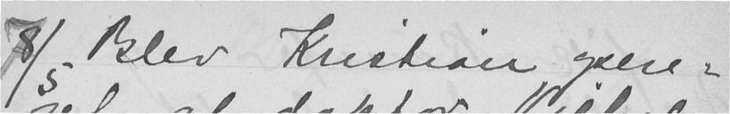8/5 Blev Kristian opere-
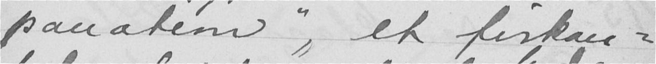panation", et firkan-
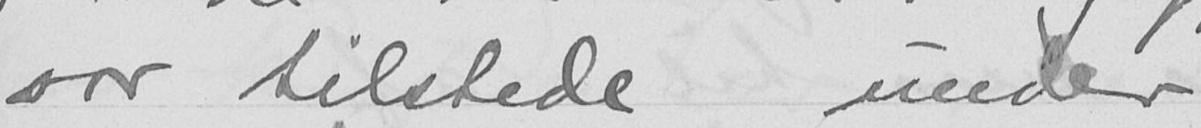var tilstede under
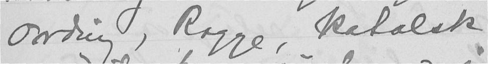Ording, Rogge, katolsk
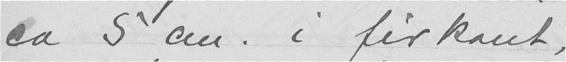ca 5 cm. i firkant,
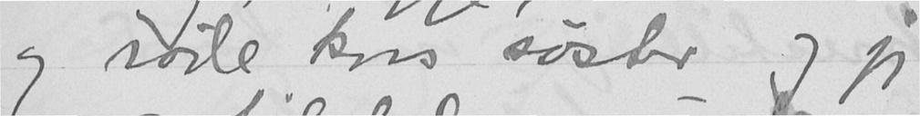og røde kors søster og jeg
